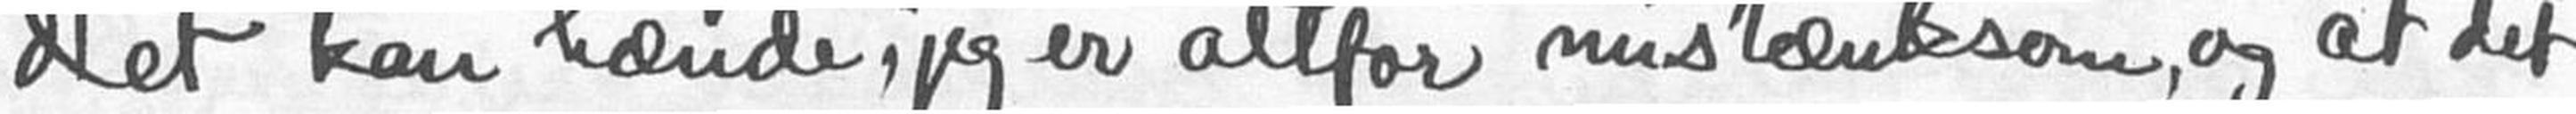det kan hænde, jeg er altfor mistænksom, og at det
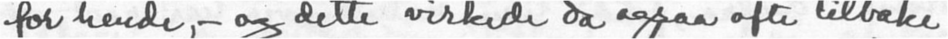for hende, - og dette virkede da ogsaa ofte tilbake,
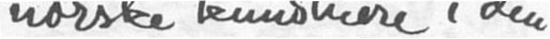norske kunstnere i den
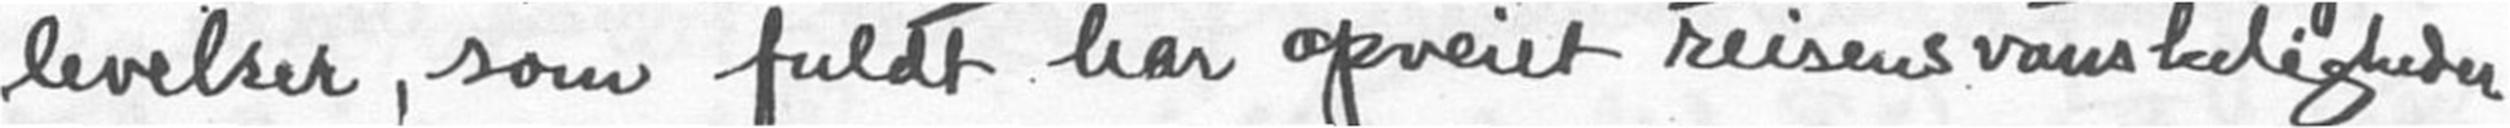levelser, som fuldt har opveiet reisens vanskeligheder.
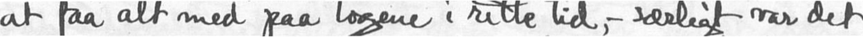at faa alt med paa togene i rette tid, - særligt var det
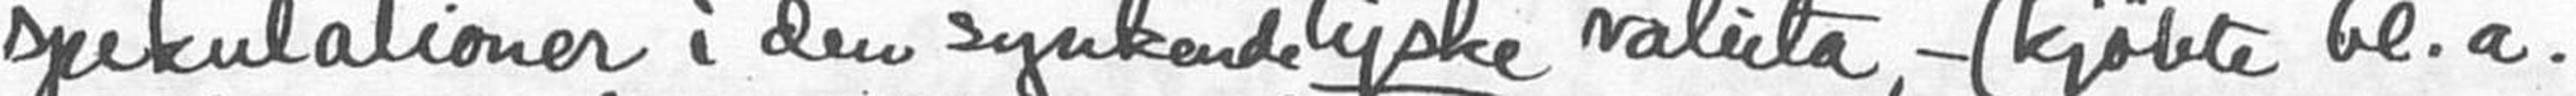spekulationer i den synkende tyske valuta, - (kjøbte bl. a.
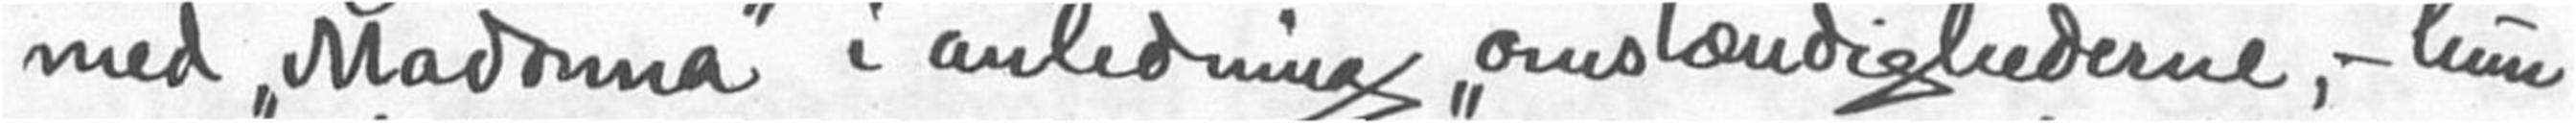med "Madonna" i anledning "omstændighederne", - hun
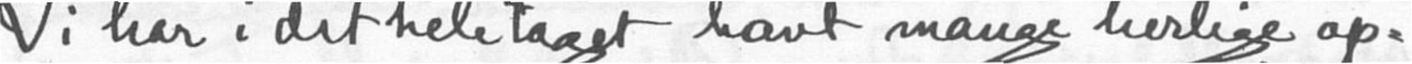Vi har i det hele taget havt mange herlige op-
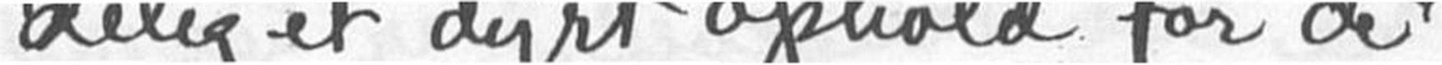delig et dyrt ophold for de
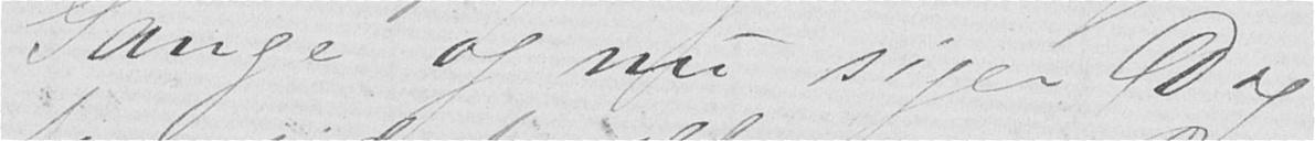Gange og nu siger Dig
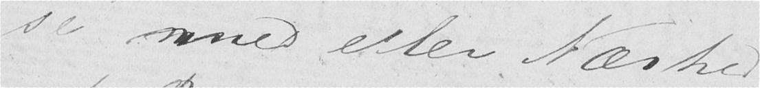se med eller Nærhed
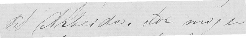til Arbeide. For mig er
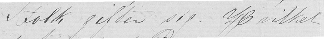Folk gifter sig. Hvilket
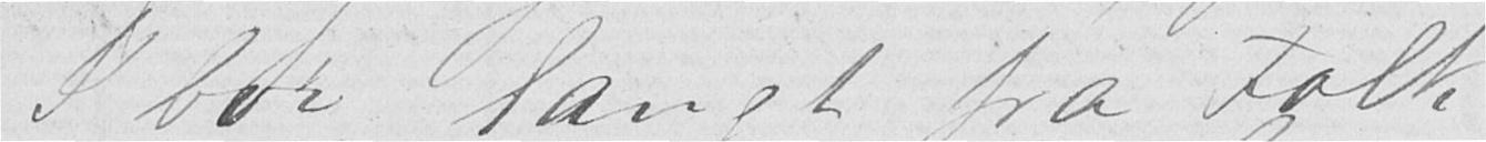i bor langt fra Folk,
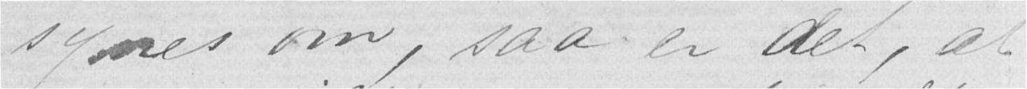synes om, saa er det, at
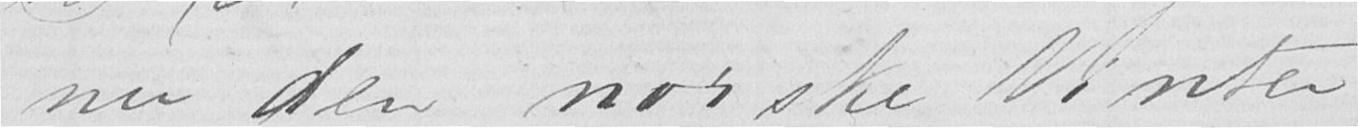nu den norske Vinter
